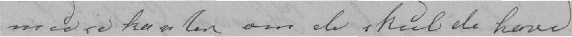musikanter om de skulde have
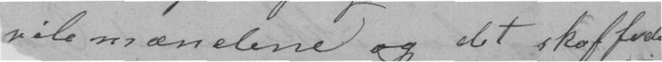spilemændene og det skaffede
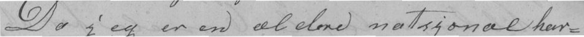Da jeg er en ældre natsjonal har-
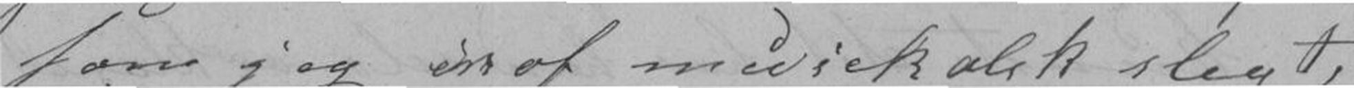som jeg er af musikalsk slegt,
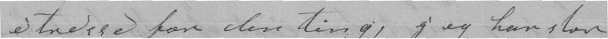itresse for den ting, jeg har stor
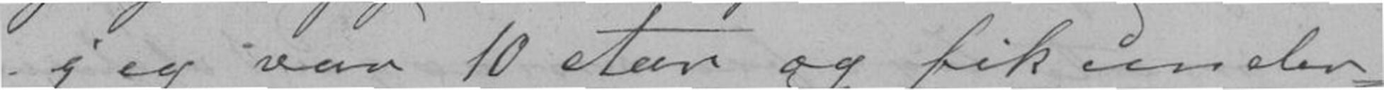jeg var 10 Aar og fik under-
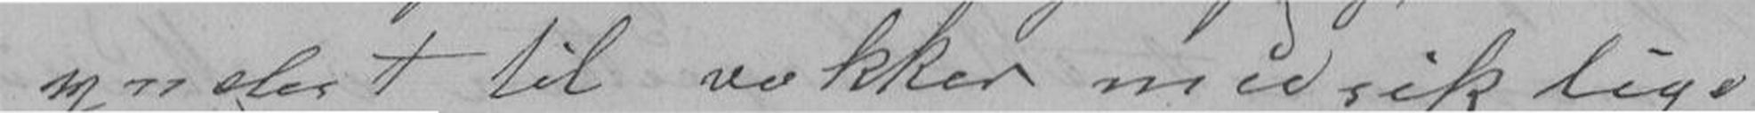yndest til vakker musik lige
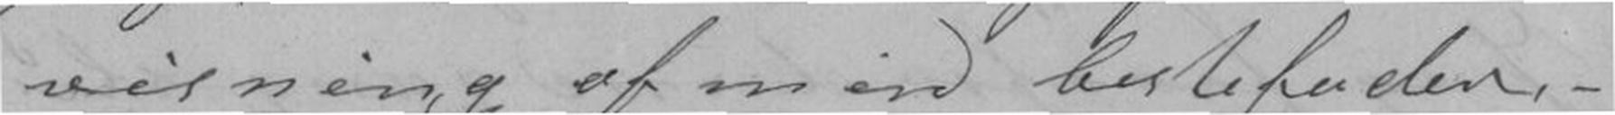visning af min bestefader, -
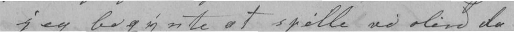jeg begynte at spille violin da
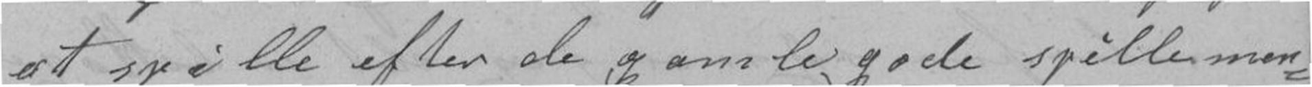at spille efter de gamle gode spillemen-
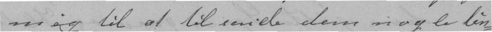mig til at tilsende dem nogle lin-
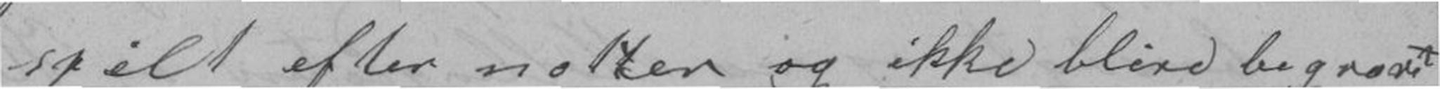spilt efter noter og ikke blive begravet
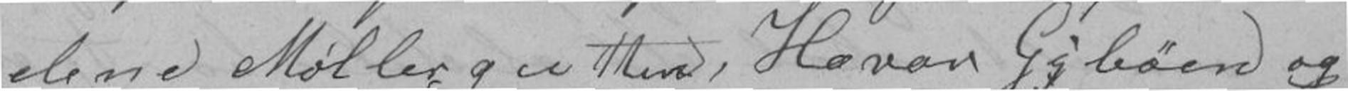dene Møllergutten, Havar Gibøen og
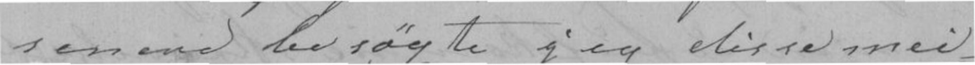senere besøgte jeg disse mei-
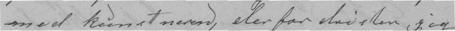med kunstneren, derfor drister jeg
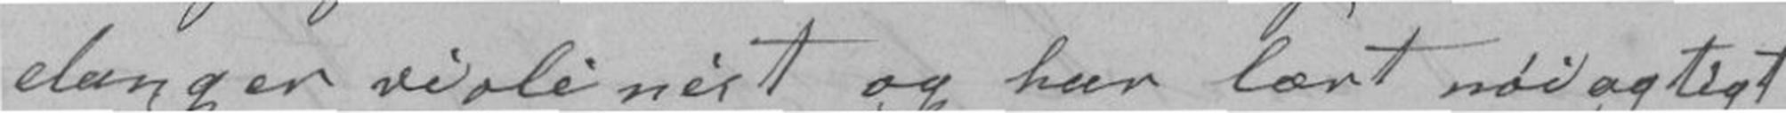danger violinist og har lært nøiagtigt
